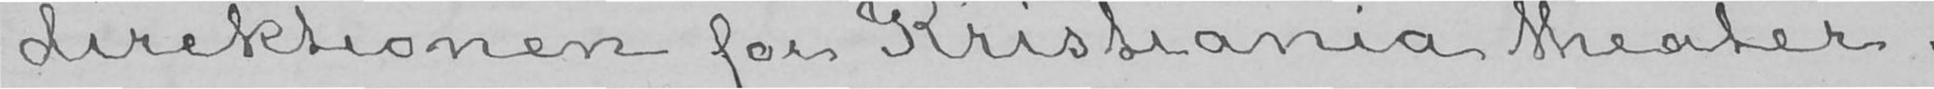direktionen for Kristiania theater.
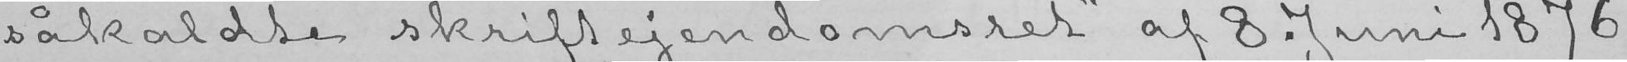såkaldte skriftejendomsret» af 8. Juni 1876,
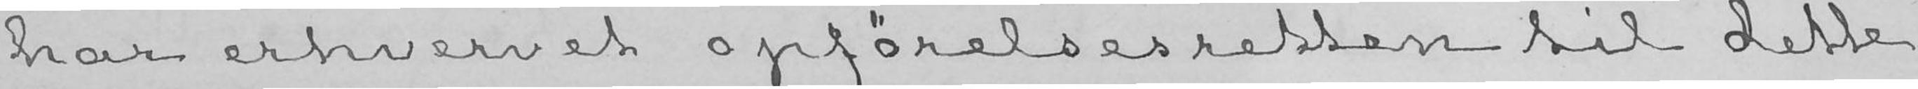har erhvervet opførelsesretten til dette
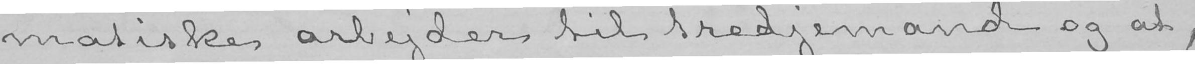matiske arbejder til tredjemand og at,
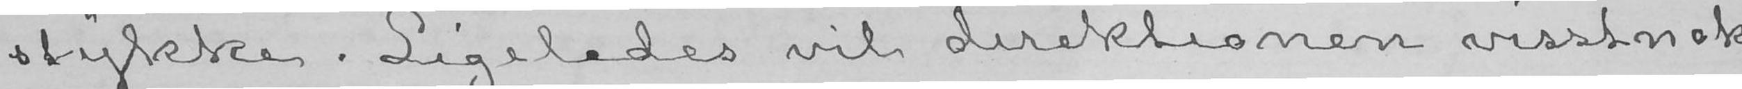stykke. Ligeledes vil direktionen visstnok
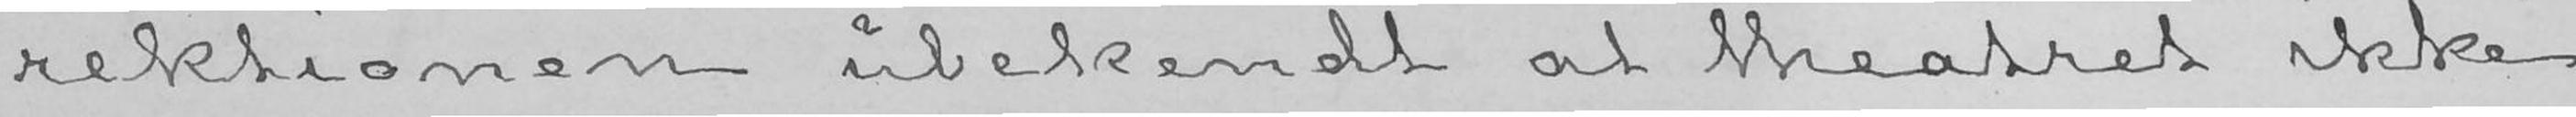rektionen ubekendt at theatret ikke
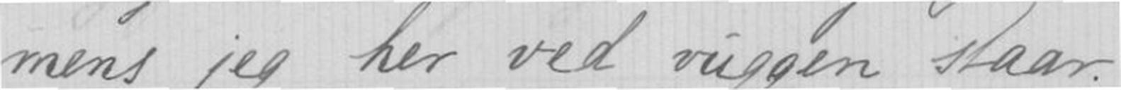mens jeg her ved vuggen staar.
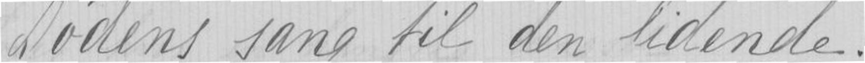Dødens sang til den lidende.
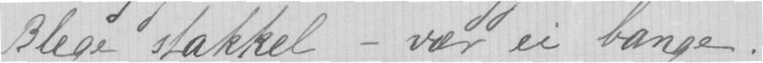Blege stakkel - vær ei bange.
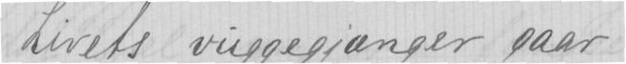Livets vuggegjænger gaar
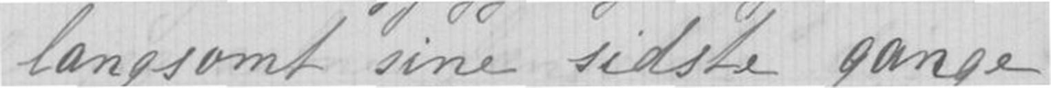langsomt sine sidste gange,
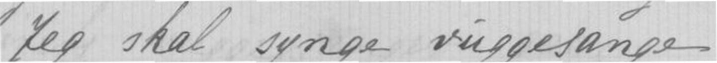Jeg skal synge vuggesange
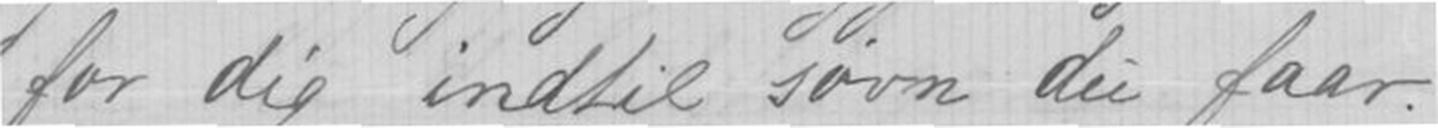for dig indtil søvn du faar.
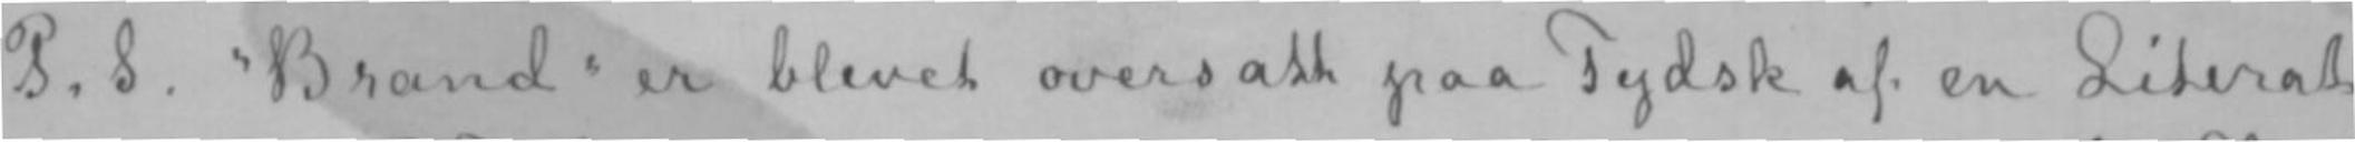P. S. «Brand» er blevet oversatt paa Tydsk af en Literat
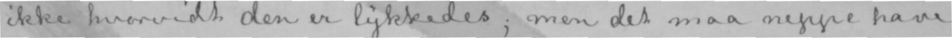ikke hvorvidt den er lykkedes; men det maa neppe have
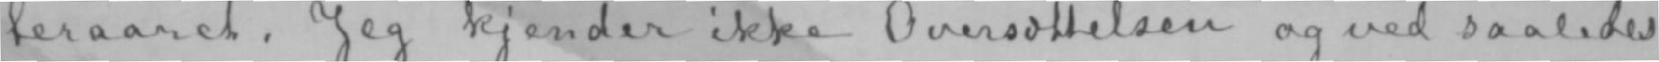teraaret. Jeg kjender ikke Oversættelsen og ved saaledes
